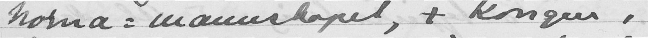Norna-manskapet, + Kongen,
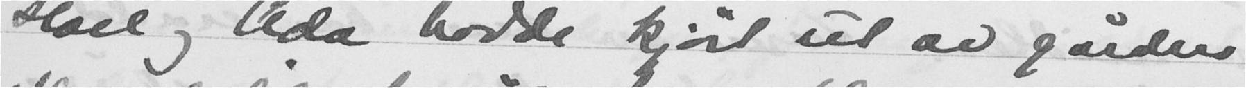Hoel og Oda hadde kjørt ut av gården
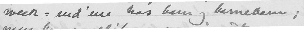week-end'ene hos barn og barnebarn;
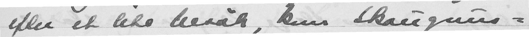efter et lite besøk, kom Skaugums-
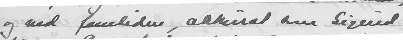og ved femtiden, akkurat som Sigurd
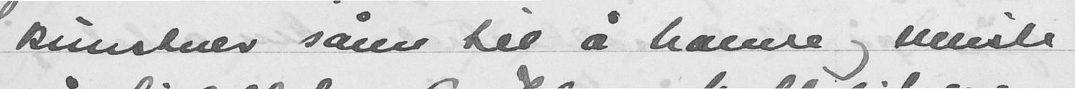kunstner sånn til å hamre og meisle
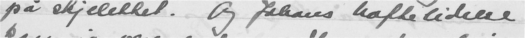på skjelettet. Og Johans hoftelidelse
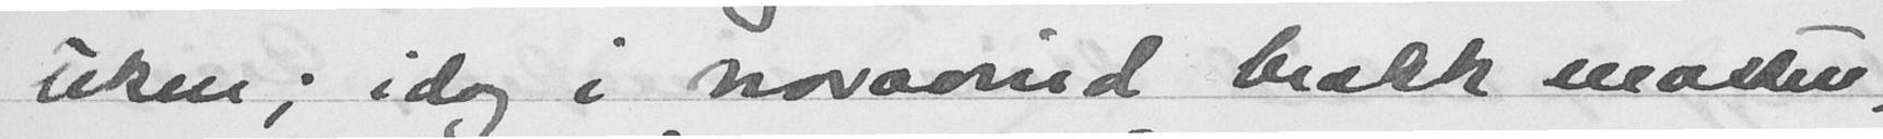uken; idag i noravind brakk masten
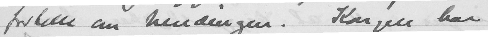fortelle om hendingen. - Kongen har
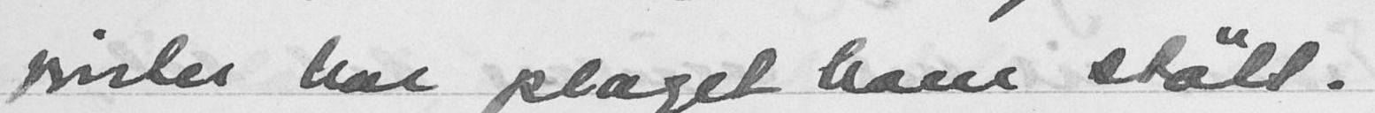vitner har plaget ham støtt.
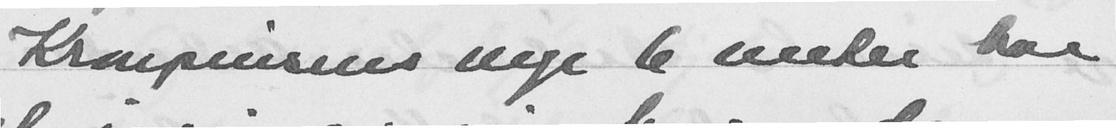Kronprinsens nye 6 meter har
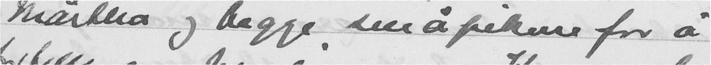Märtha og begge småpiken for å
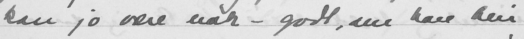kan jo være nok - godt, om han blir
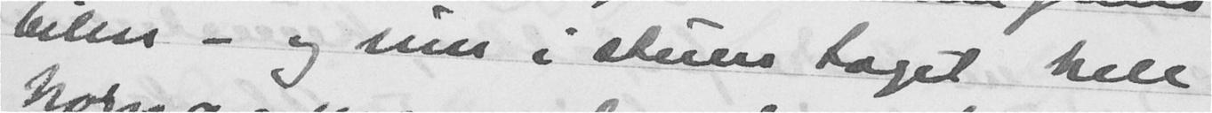bilen - og inn i stuen laget hele
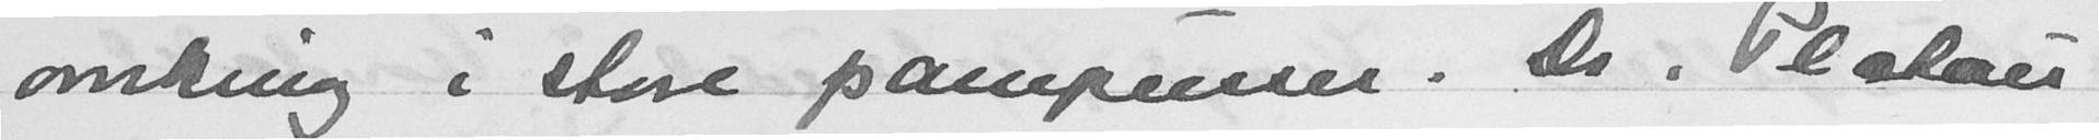omkring i store pampusser. Dr. Platau,
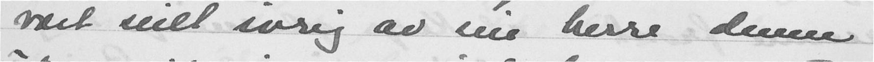vært seilt ivrig av sin herre denne
